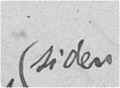siden
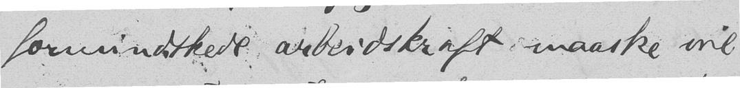formindskede arbeidskraft maaske vil
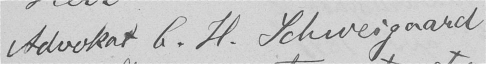Advokat C. H. Schweigaard
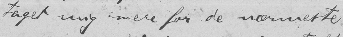taget mig mere for de nærmeste
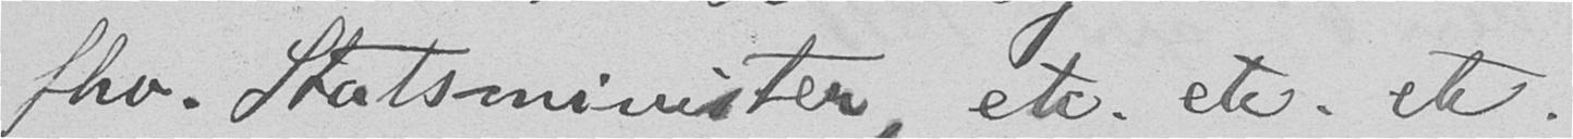fhv. Statsminister, etc. etc. etc.
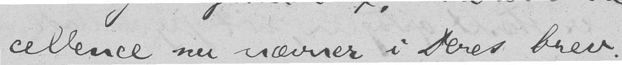cellence nu nævner i Deres brev.
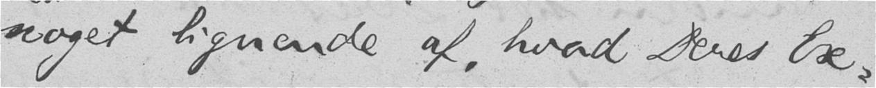noget lignende af, hvad Deres Ex-
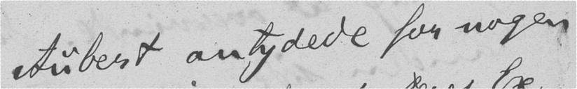Aubert antydede for nogen
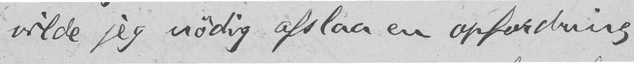vilde jeg nødig afslaa en opfordring
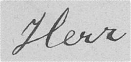Herr
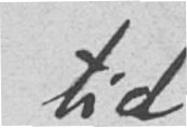tid
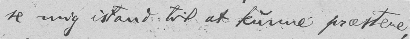se mig istand til at kunne præstere,
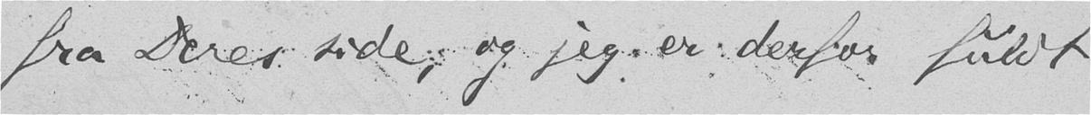fra Deres side, og jeg er derfor fuldt
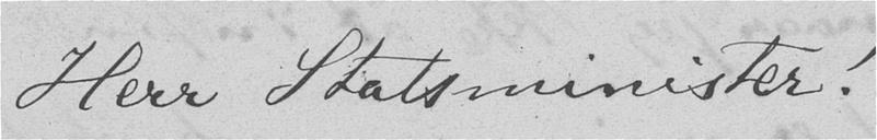Herr Statsminister!
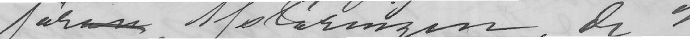føran Afsløringen, de 3
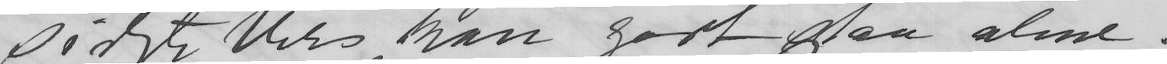sidste Vers kan godt staa alene.
